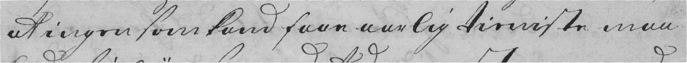at ingen som kand faae aarlig tieniste maa
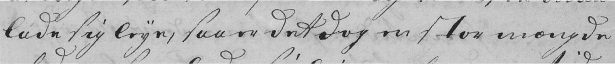lade sig leye, saa er det dog en stor mængde
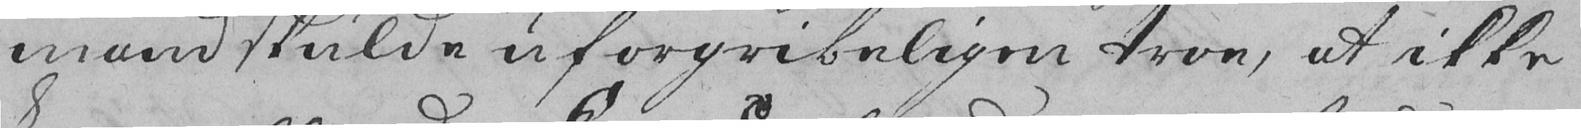mand skulde uforgribeligen troe, at ikke
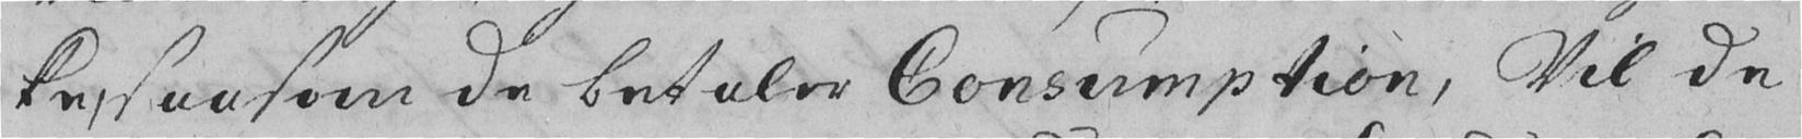ke, saasom de betaler Consumption, Vil de
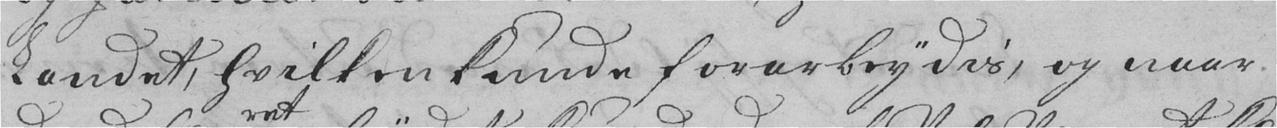Landet, hvilken kunde forarbeydis, og naar
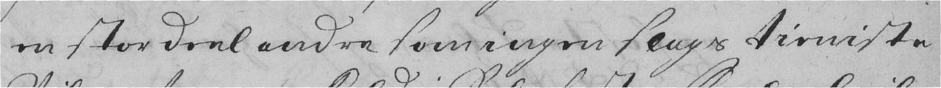en stor deel andre som ingen slags tieniste
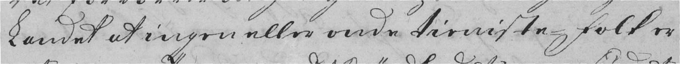Landet at ingen eller onde tieniste-Folk er
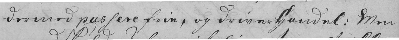dermed passere frie, og driver Handel. Men
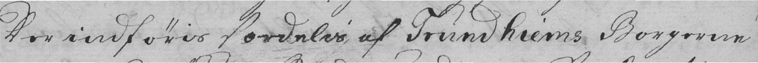Der indføris særdelis af Trundhiems Borgerne
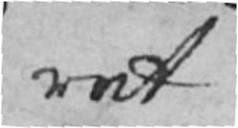ret
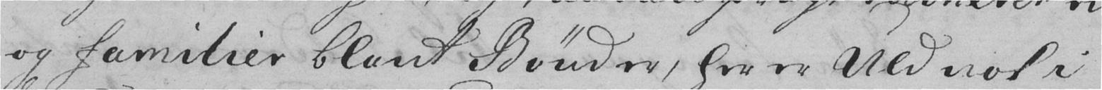og familier blant Bønder, her er Uld nok i
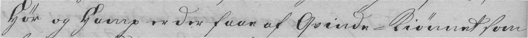Hør og Hamp er der faae af Qvinde-Kiønnet som
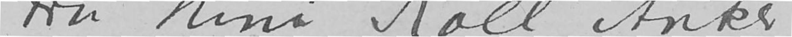Fru Nini Roll Anker
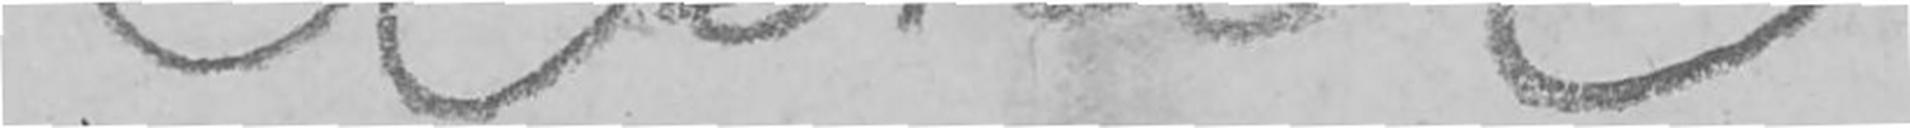Asker
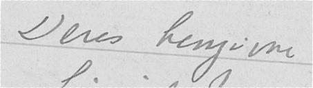Deres hengivne
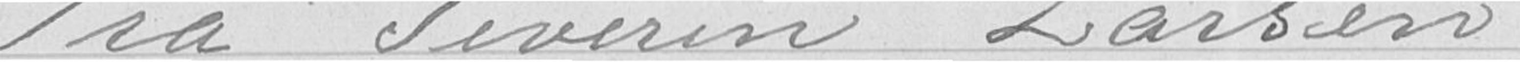Fra Severin Larsen
